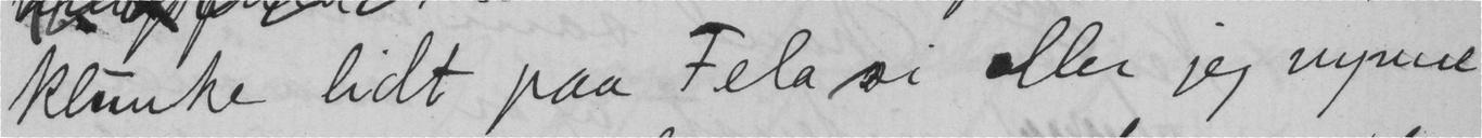klunke lidt paa Fela si eller jeg nynne
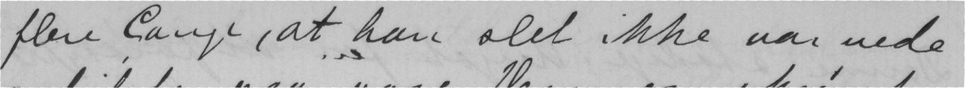flere Gange, at han slet ikke var nede
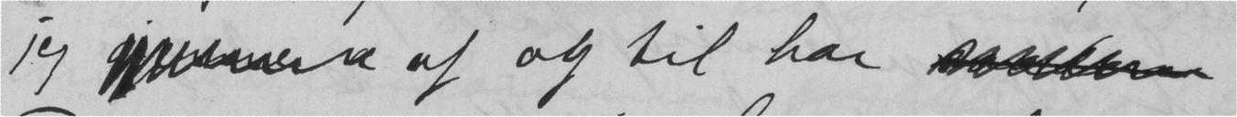jeg  af og til har
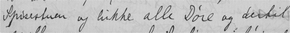Spisestuen og lukke alle Døre og dertil
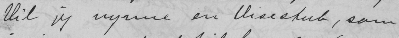Vil jeg nynne en Visestub, som
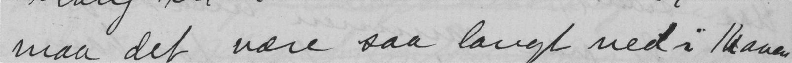maa det være saa langt ned i maven
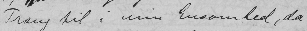Trang til i min Ensomhed, da
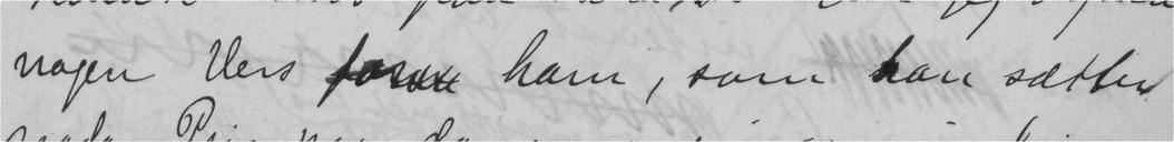nogen Vers for ham, som han sætter
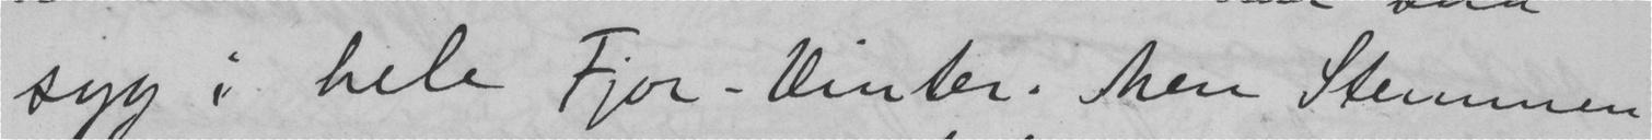syg i hele Fjor-Vinter. Men Stemmen
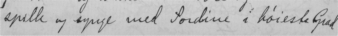spille og synge med Sordine i høieste Grad
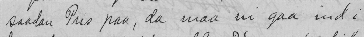saadan Pris paa, da maa vi gaa ind i
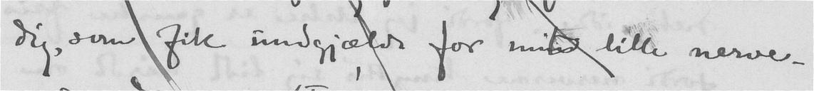dig, som fik undgjælde for mit lille nerve-
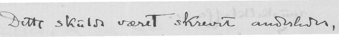Dette skulde været skrevet anderledes,
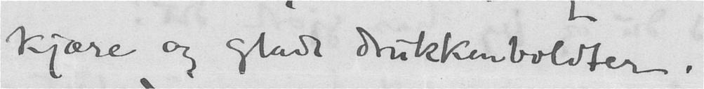kjære og glade drukkenboldter.
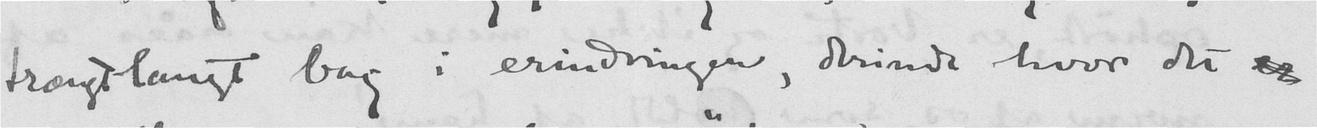trængt langt bag i erindringen, derinde hvor det er
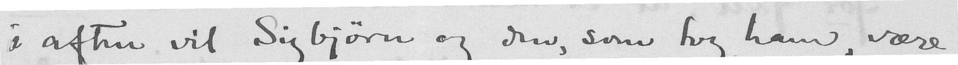i aften vil Sigbjørn og den, som tog ham, være
